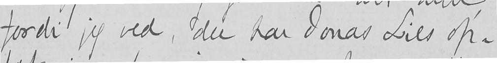fordi jeg ved, du har Jonas Lies op-
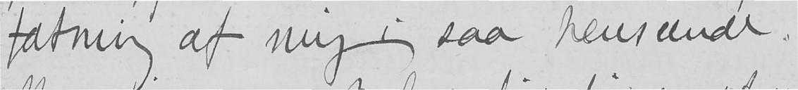fatning af mig i saa henseende.
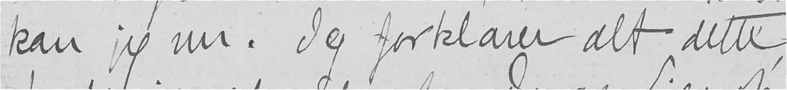kan jeg nu. Jeg forklarer alt dette,
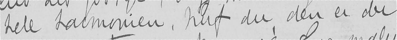hele harmonien, huf du den er der
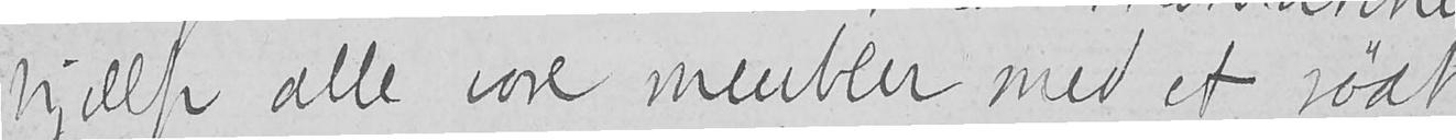hjælp alle vore meubler med et rødt
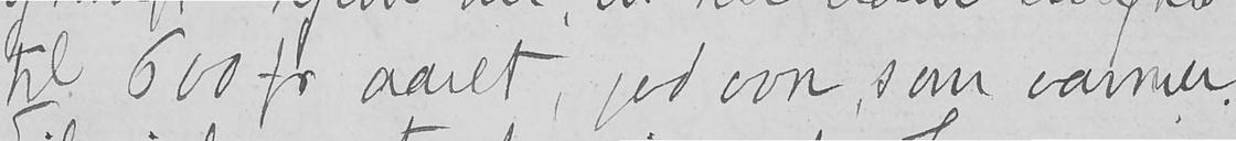til 600 for aaret, vedovn, som varmer.
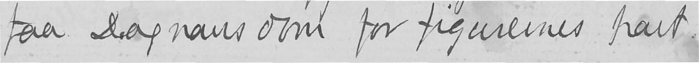faa Dagnans dom for figurernes part.
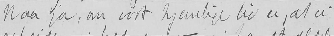Naa ja, om vort hjemlige liv er, at vi
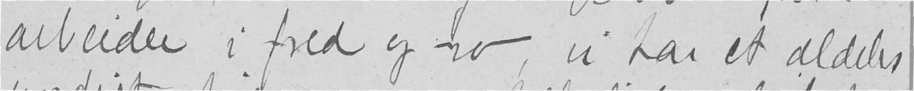arbeider i fred og ro, vi har et aldeles
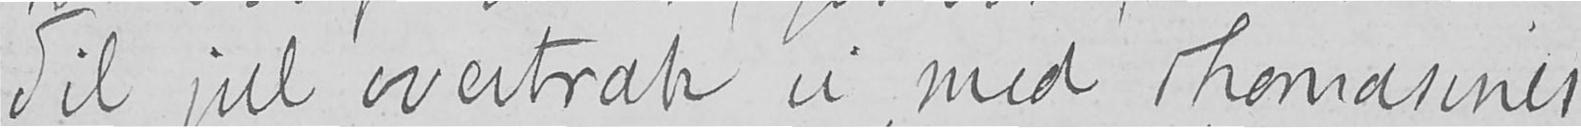Til jul overtrak vi med Thomasines
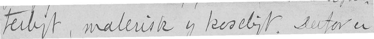terligt, malerisk og koseligt. Derfor er
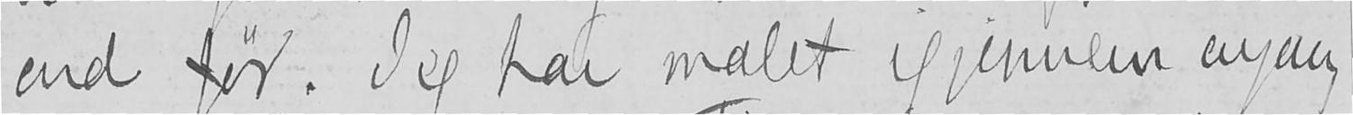end før. Jeg har malet igjennem engang
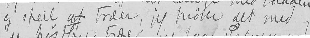og speil af træer, jeg prøver det med
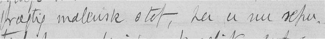prægtig malerisk stof, her er nu repu-
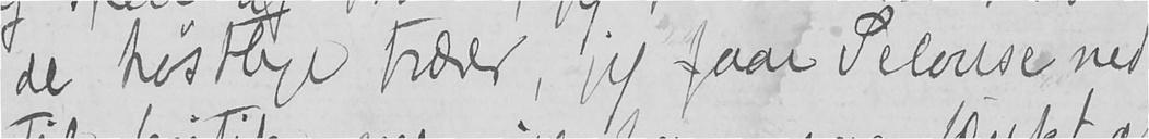de høstlige træer, jeg faar Pelouse ned
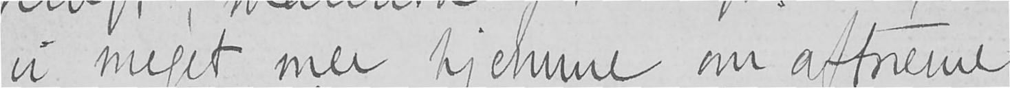vi meget mer hjemme om aftnerne
